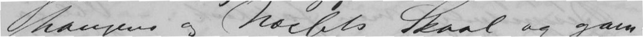haugens og Næssets Skaal og gam-
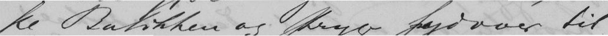ke Butikken og stryge sydover til
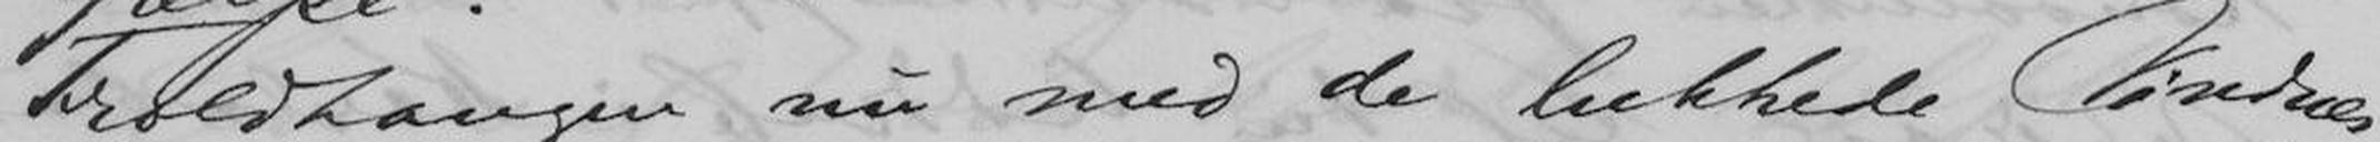Troldhaugen nu med de lukkede Vindues-
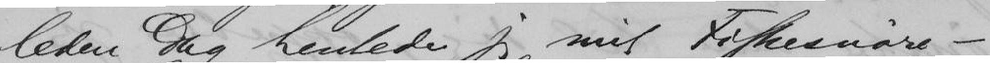leden Dag hentede jeg mit Fiskesnøre -
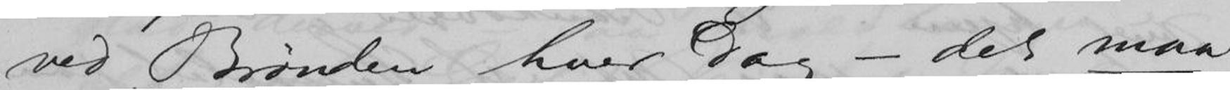ved Brønden hver Dag - det maa
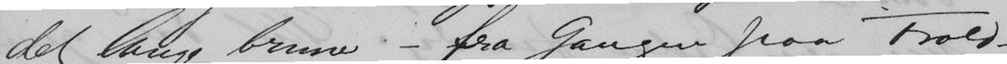det lange brune - fra Gangen paa Trold-
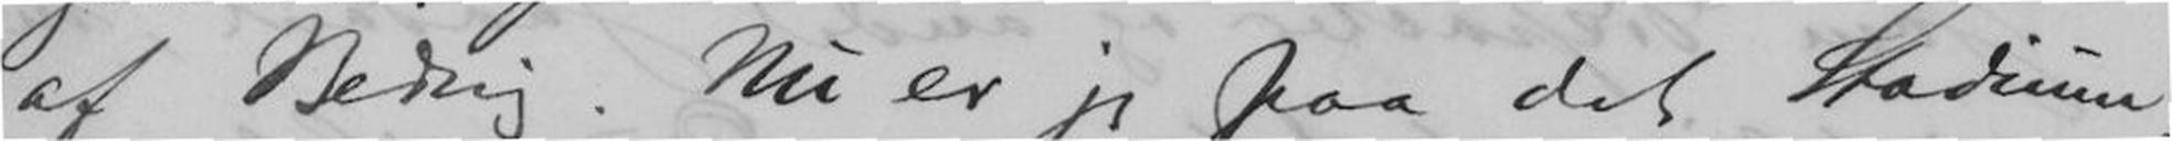af Bedring. Nu er jeg paa det Stadium,
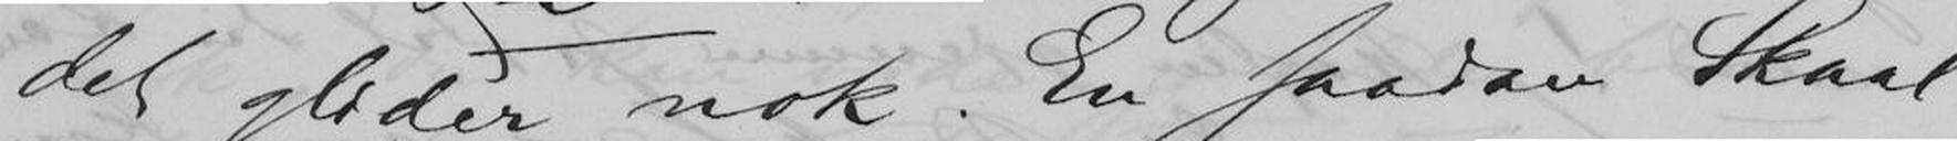det glider nok. En saadan Skaal
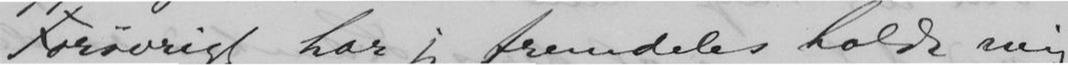Forøvrigt har jeg fremdeles holdt mig
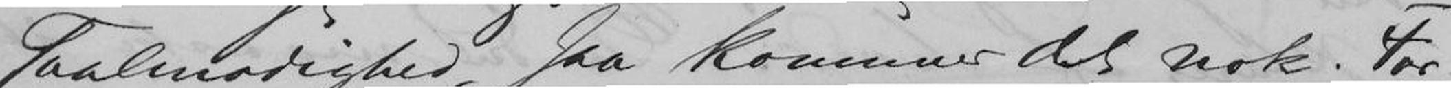Taalmodighed, saa kommer det nok. For-
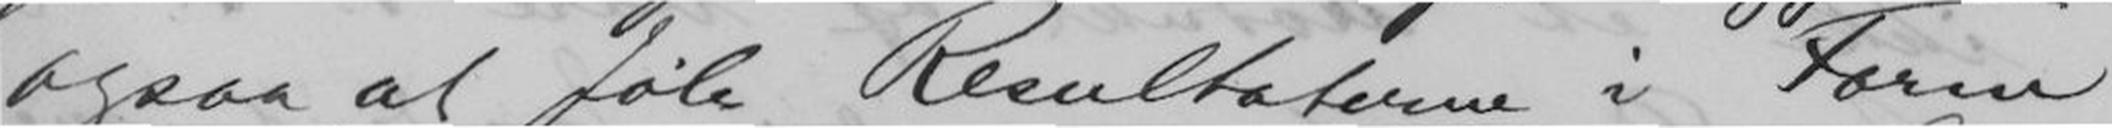ogsaa at føle Resultaterne i Form
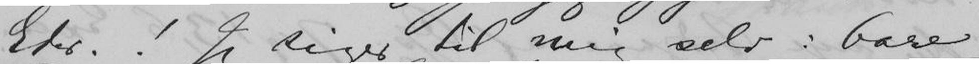Eder. ! Jeg siger til mig selv: bare
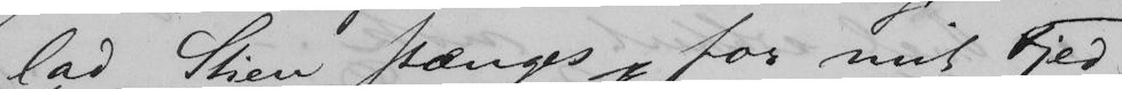- lad Stien stænges for mit Fjed,
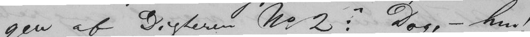gen af Digteren N. 2: "Dog - hm!
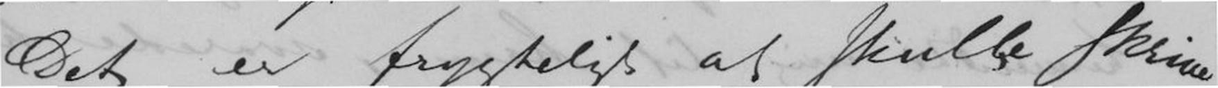Det er frygteligt at skulle skrive
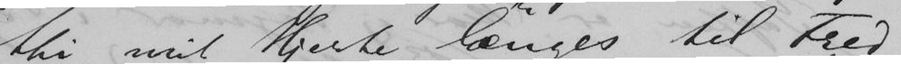thi mit Hjerte længes til Fred"!
